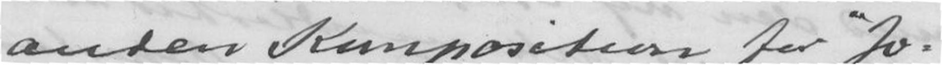anden Komposition for "Jo-
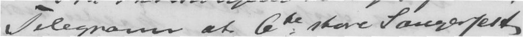Telegram at 6te store Sangerfest
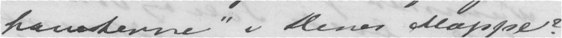haniterne" i Deres Mappe?
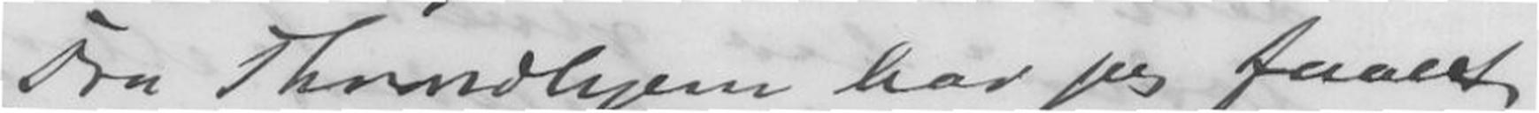Fra Thromdhjem har jeg faaet
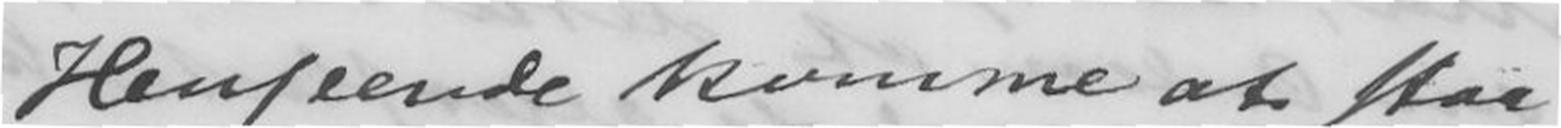Henseende komme at staa
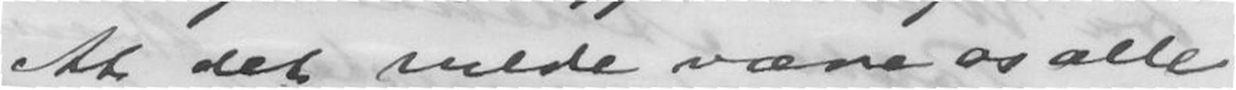At det vilde være os alle
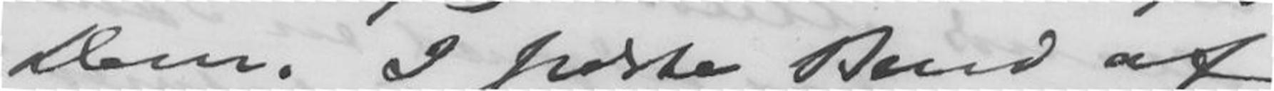Dem. I sidste Bind af
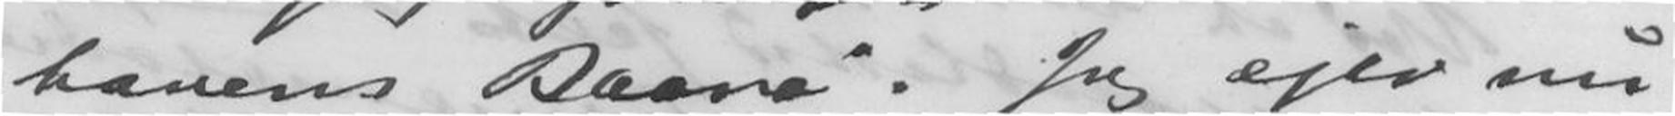havens Baare". Jeg ejer nu
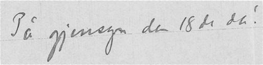På gjensyn den 18de da!
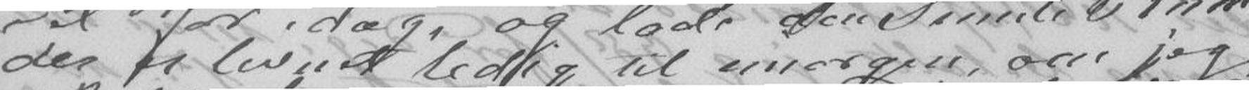der er levnet ledig til imorgen, om jeg
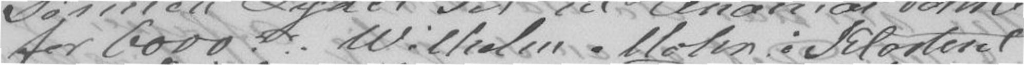for 6000do. Wilhelm Mohr i Klosteret
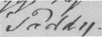Paddy.
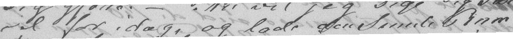vel for idag, og lade een Smule Rum
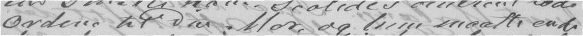Ordene til Din Mor, og hun maatte end-
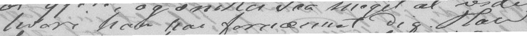hvor han har fornærmet Dig. Han
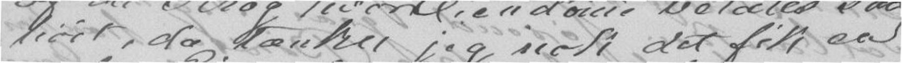høit, da tænker jeg nok det fik en
